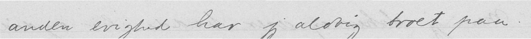anden evighed har jeg aldrig troet paa.
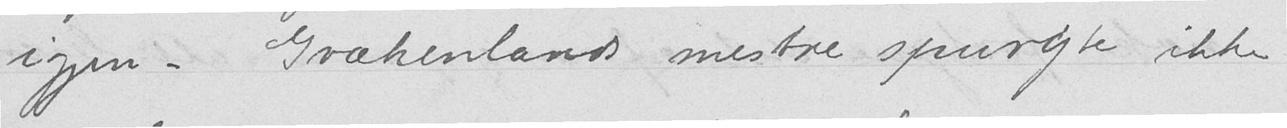igjen. Grækenlands mestre spurgte ikke
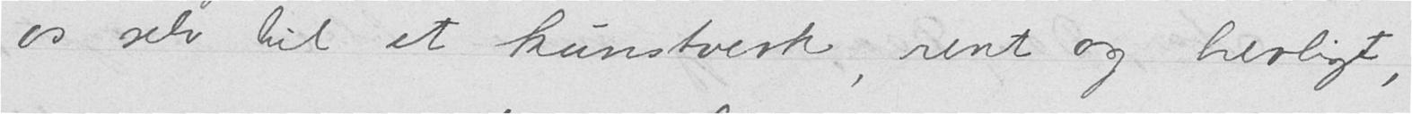os selv til et kunstverk, rent og herligt,
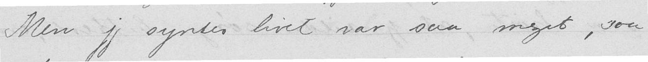Men jeg syntes livet var saa meget, saa
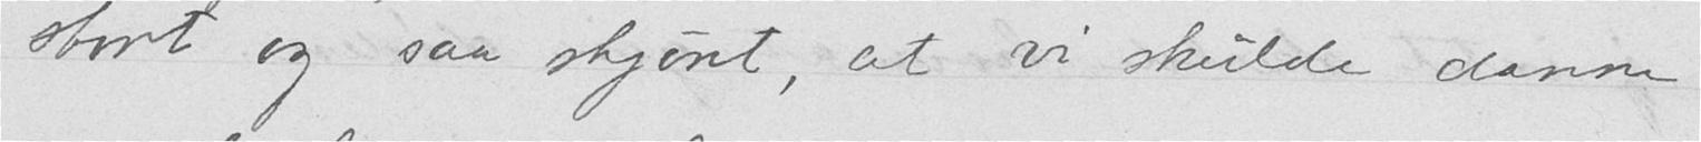stort og saa skjønt, at vi skulde danne
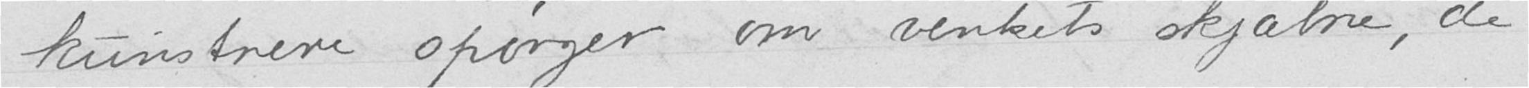kunstnere spørger om verkets skjæbne, de
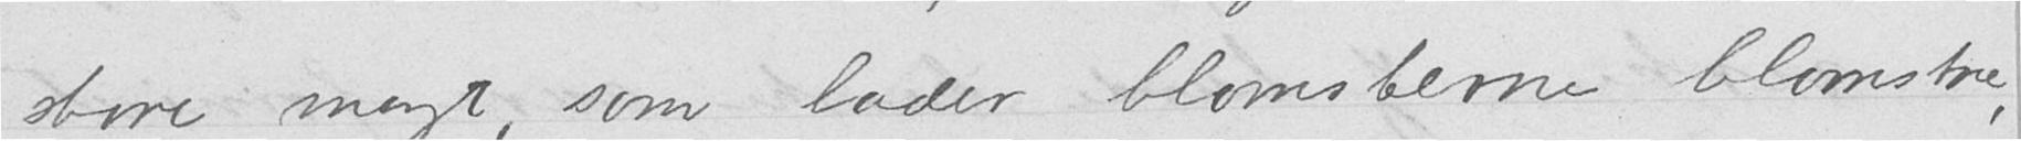store magt, som lader blomsterne blomstre,
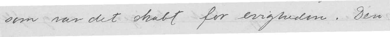som var det skabt for evigheden. Den
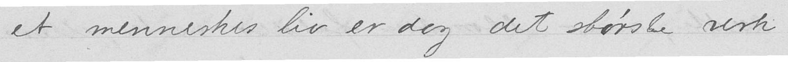et menneskes liv er dog det største verk
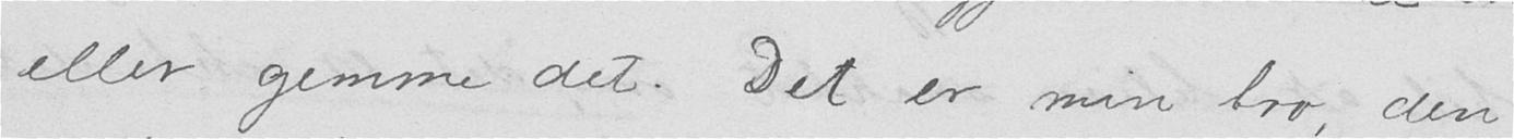eller gemme det. Det er min tro, den
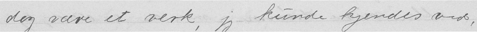dog være et verk, jeg kunde kjendes ved,
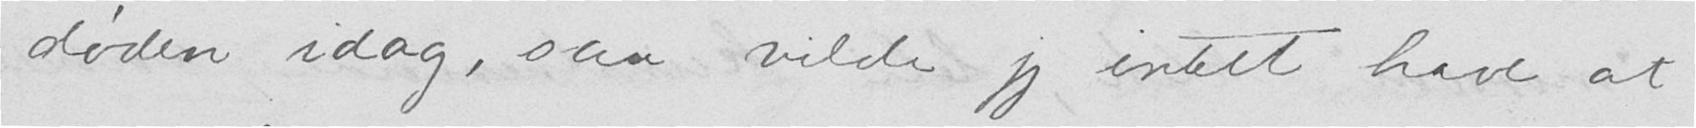døden idag, saa vilde jeg intet have at
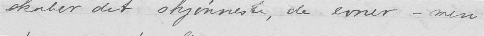skaber det skjønneste, de evner - men
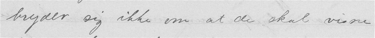bryder sig ikke om at de skal visne
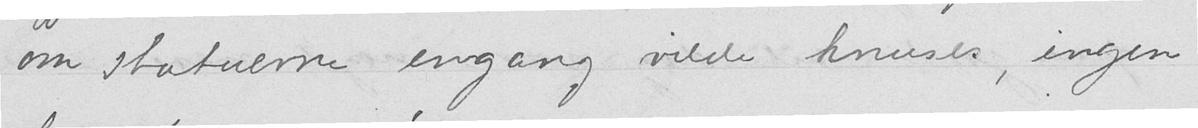om statuerne engang vilde knuses, ingen
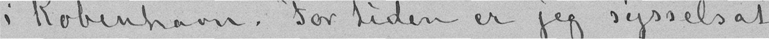i København. For tiden er jeg sysselsat
